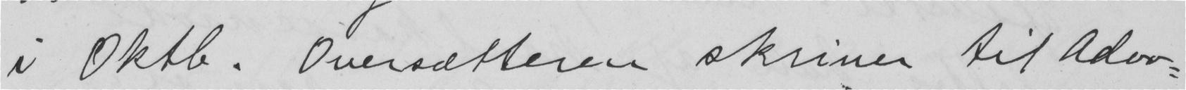i Oktb. Oversætteren skriver til Advo-
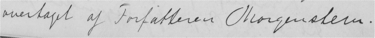overtaget af Forfatteren Morgenstern.
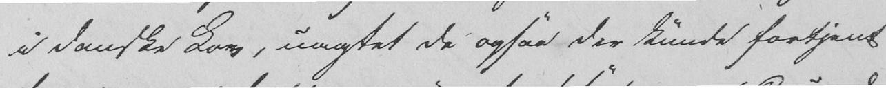i danske Lov, uagtet de ogsaa der kunde fortjent
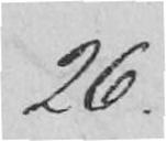26.
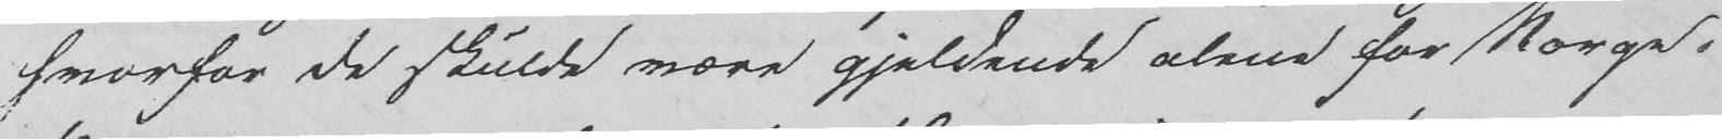hvorfor de skulde være gjeldende alene for Norge.
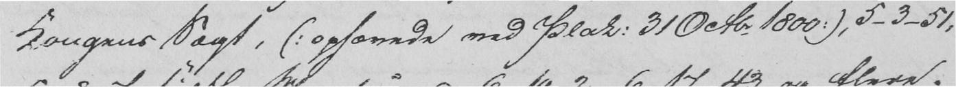Kongens Sægt, (: ophævede ved Plak. 31 Octbr 1800 :), 5-3-51,
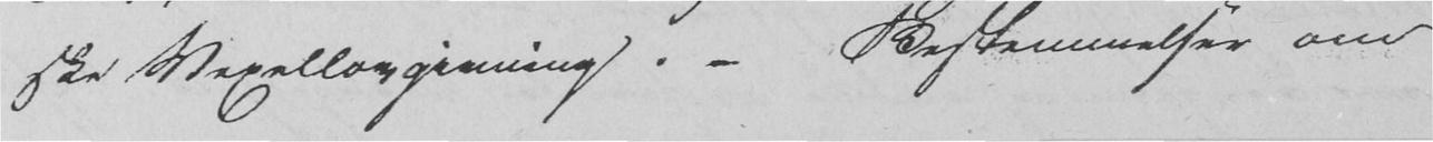ske Vexellovgivning. - Bestemmelser om
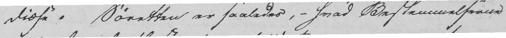disse. Søretten er saaledes, - hvad Bestemmelserne
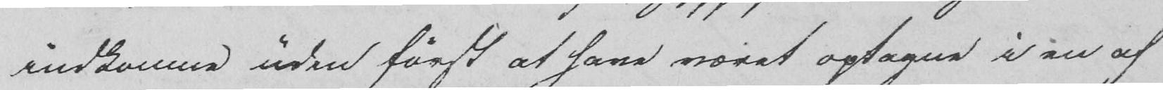indkomne uden først at have været optagne i en af
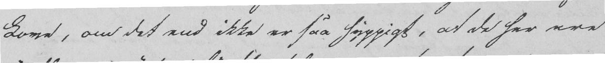Love, om det end ikke er saa hyppigt, at de her ere
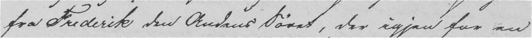fra Frederik den Andens Søret, der igjen for en
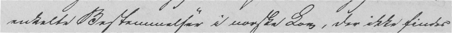enkelte Bestemmelser i norske Lov, der ikke findes
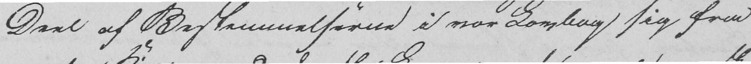Deel af Bestemmelserne i vor Lovbog sig fra
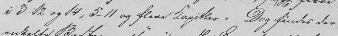i 3-12 og 14, 5-11 og flere Kapitler. Dog findes der
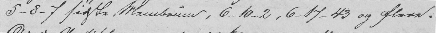5-8-7 sidste Membrum, 6-10-2, 6-17-43 og flere.
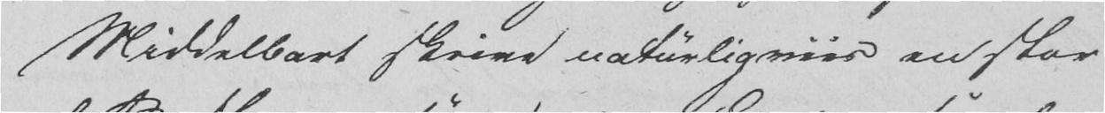Middelbart skrive naturligviis en stor
